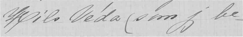Hils Veda, som jeg be-
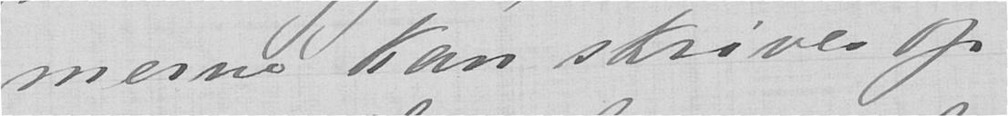merne kan skrives op
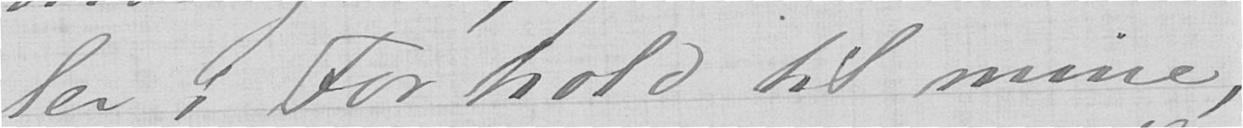ler i Forhold til mine,
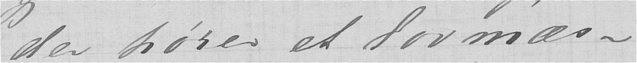der hører et lovmæs-
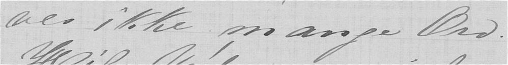ves ikke mange Ord.
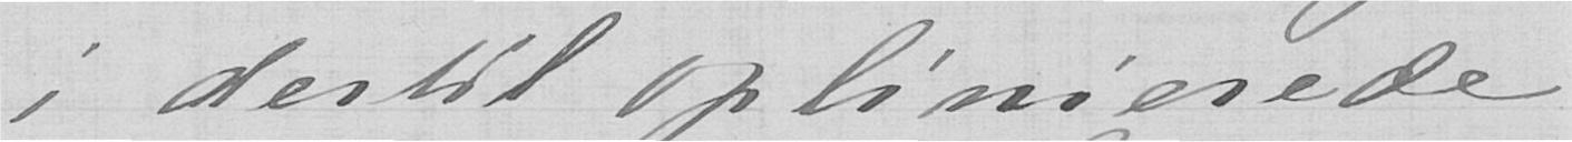i dertil oplinierede
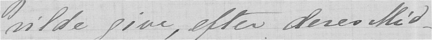vilde give, efter deres Mid-
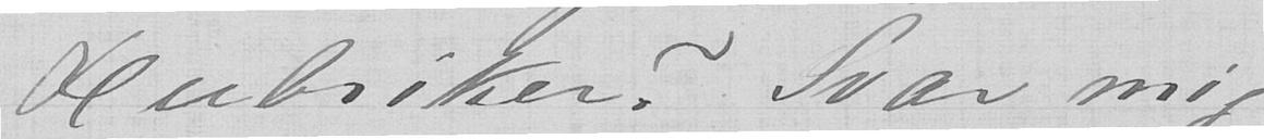Rubriker? Svar mig
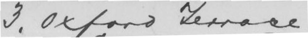3. Oxford Terrace
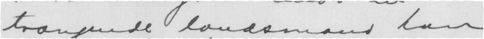trængende landsmand kan
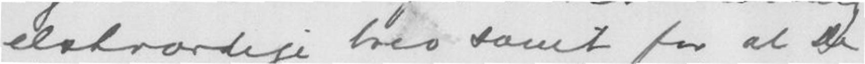elskværdige brev samt for at De
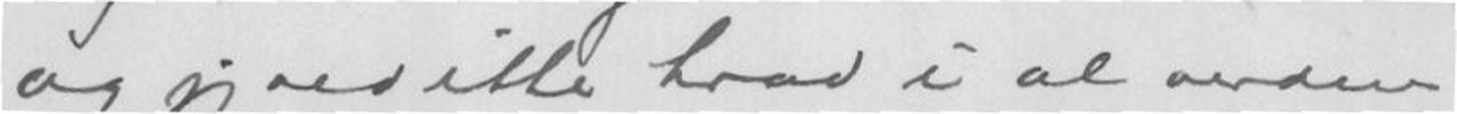og jeg ved ikke hvad i al verden
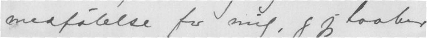medfølelse for mig, og jeg haaber
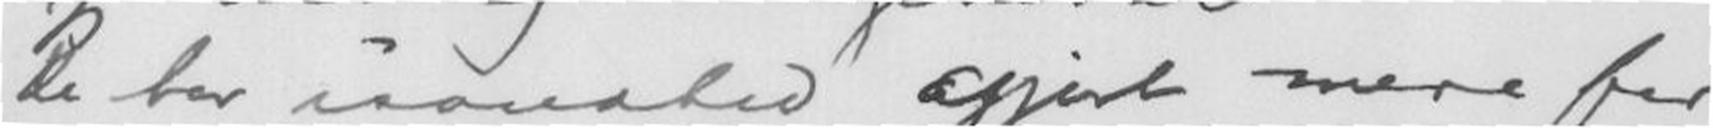De har isandhed afjort mere for
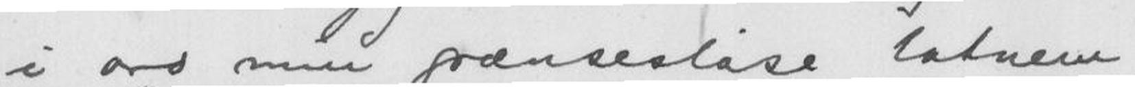i ord min grænseløse taknem-
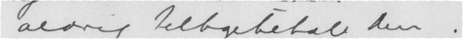jeg aldrig tilbagebetale Den.
